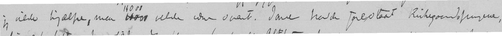jeg vilde hjælpe, men 11000 vilde være svært. Jane havde foreslaat Kirkegaardspengene,
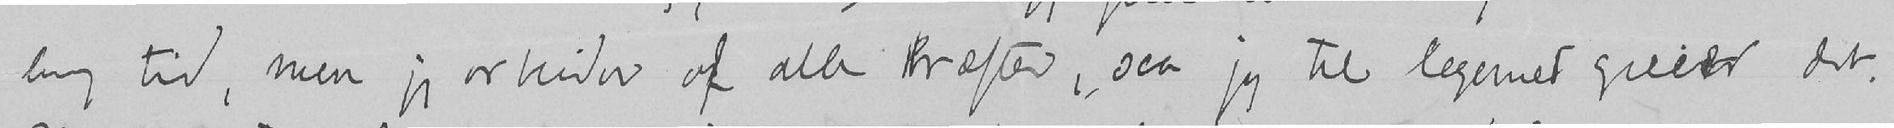lang tid, men jeg arbeider af alle kræfter, saa jeg til legemet greier det.
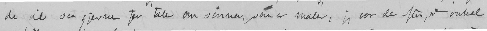de vil saa gjerne faa tale om sønnen, som er maler, jeg var der efter, at onkel
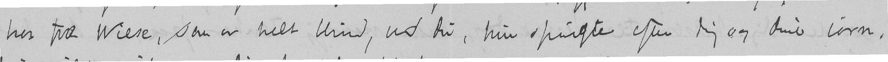hos fru Wiese, som er helt blind, ved Du, hun spurgte efter dig og dine børn,
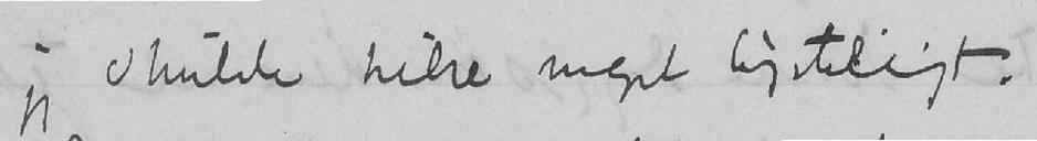jeg skulde hilse meget hjerteligt.
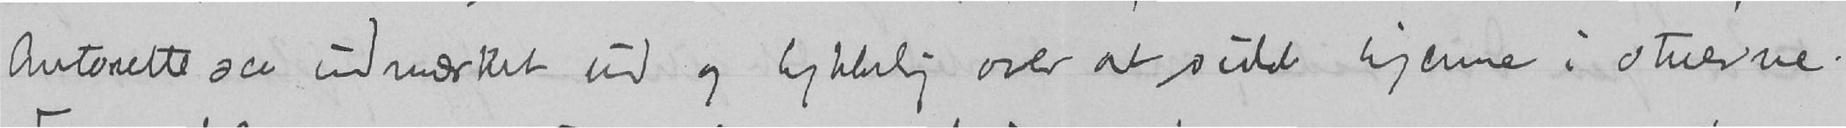Antonette saa udmærket ud og lykkelig over at sidde hjemme i stuerne.
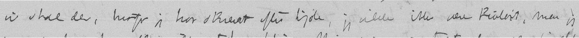vi skal der, hvorfor jeg har skrevet efter kjole, jeg vilde ikke være kulørt, men jeg
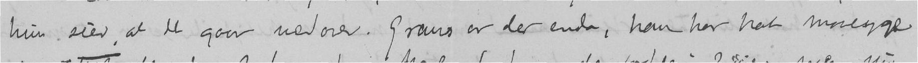hun sier, at det gaar nedover. Grans er der enda, han har hat mavesyge
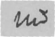nu
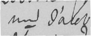med Jacob
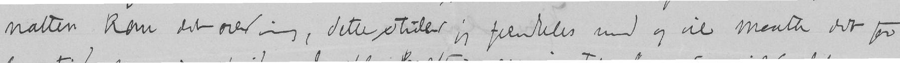natten kom det over mig, dette strider jeg fremdeles med og vil maatte det for
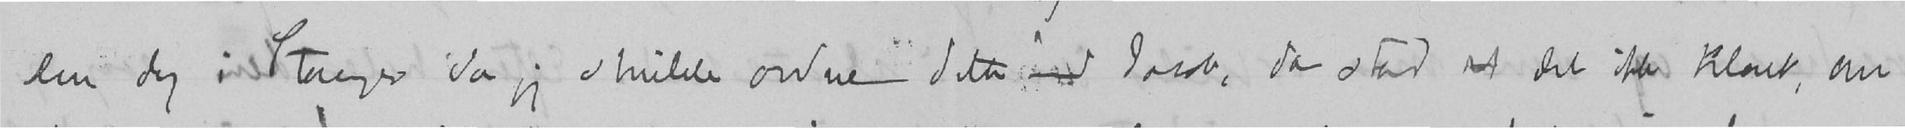Den dag i Stavanger da jeg skulde ordne dette med Jacob, da stod et det ikke klart, om
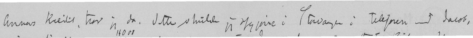Annas Kredit, tror jeg da. Dette skulde jeg afgjøre i Stavanger i telefonen med Jacob,
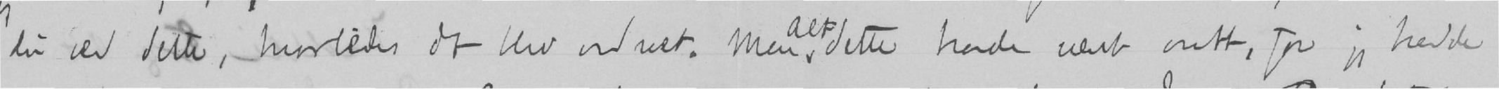du ved dette, hvorledes det blev ordnet. Men alt dette havde været ondt, for jeg havde
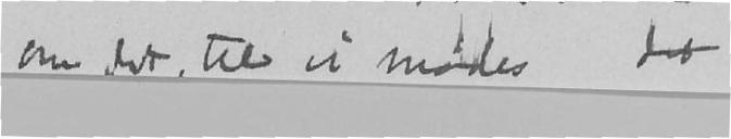om det, til vi mødes
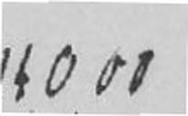14000
